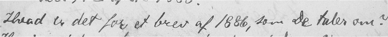Hvad er det for et brev af 1886, som De taler om?
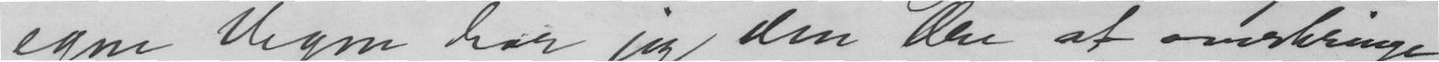egne Vegne har jeg den Ære at overbringe
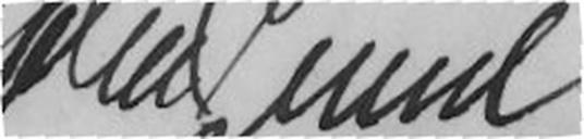John Lund
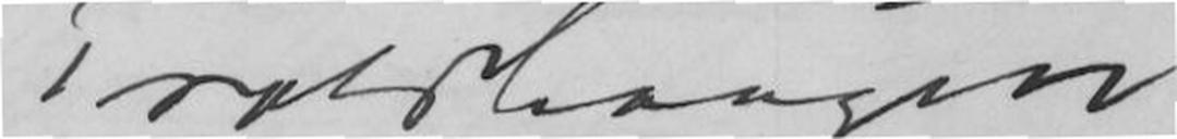Troldhaugen
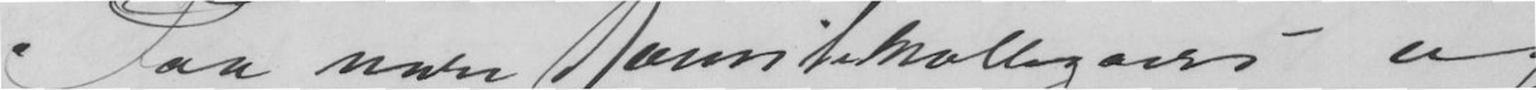Paa vore Komitekollegaes og
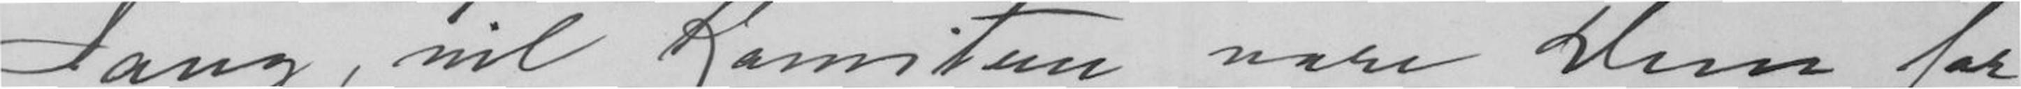Sang, vil Komiteen være Dem for-
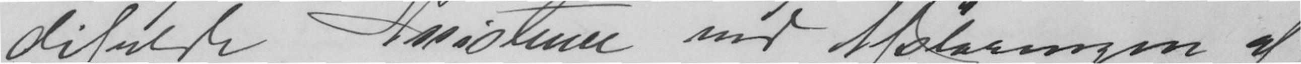difulde Assistence ved Afsløringen af
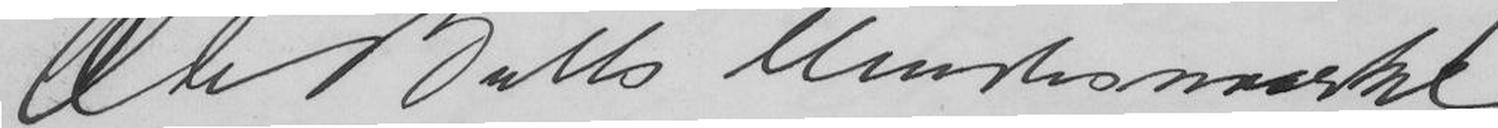Ole Bulls Mindesmerke
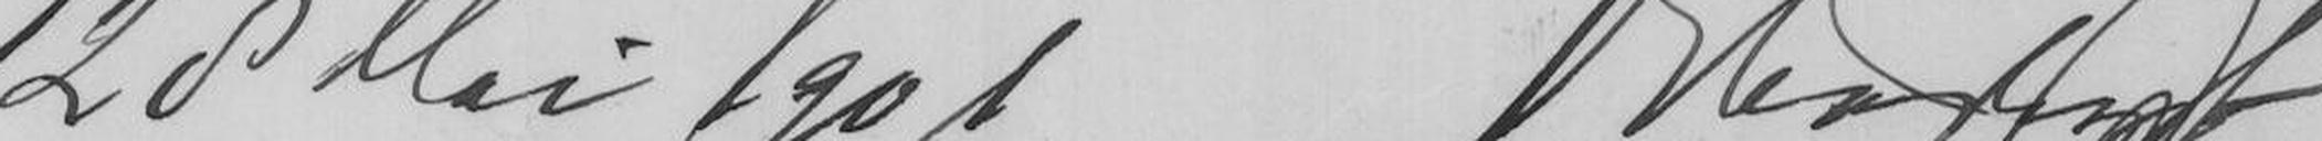28 Mai 1901 Ærbødigst
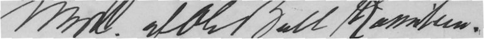Medl af Ole Bull Komiteen.
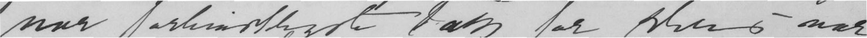vor forbintligste Tak for Deres vær-
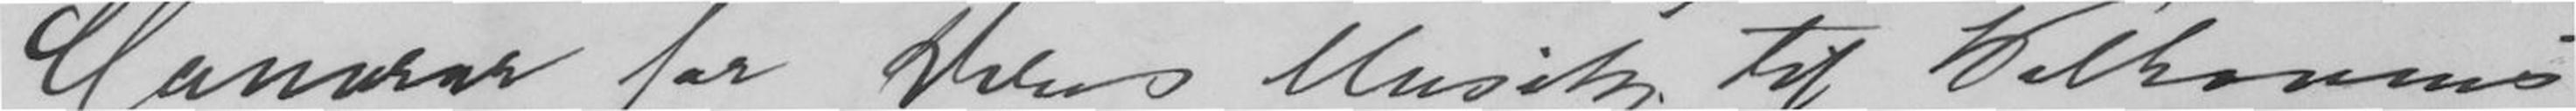Honorar for Deres Musik til Welhavens
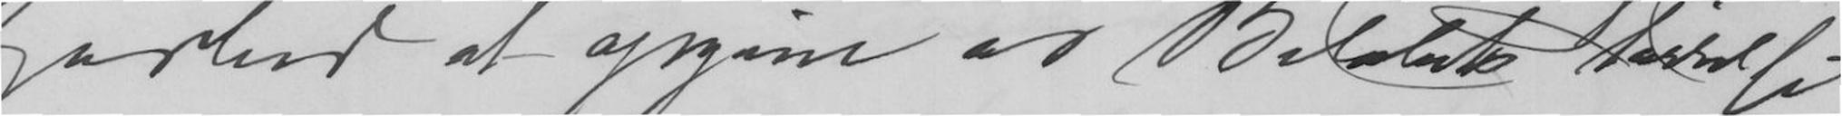Godhed at opgive os Beløbet Størrelse
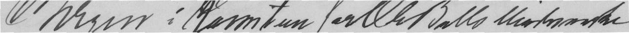Bergen: Komiteen for Ole Bulls Mindesmærke
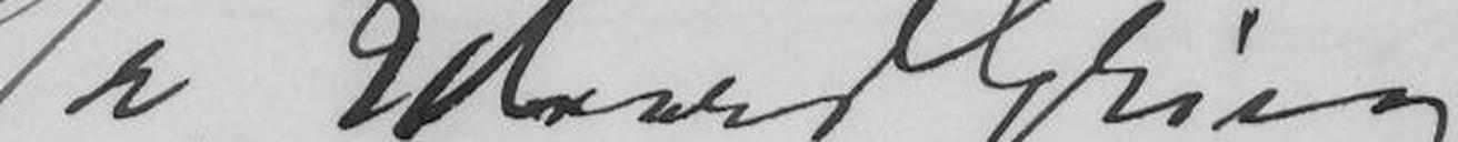Hr. Edvard Grieg
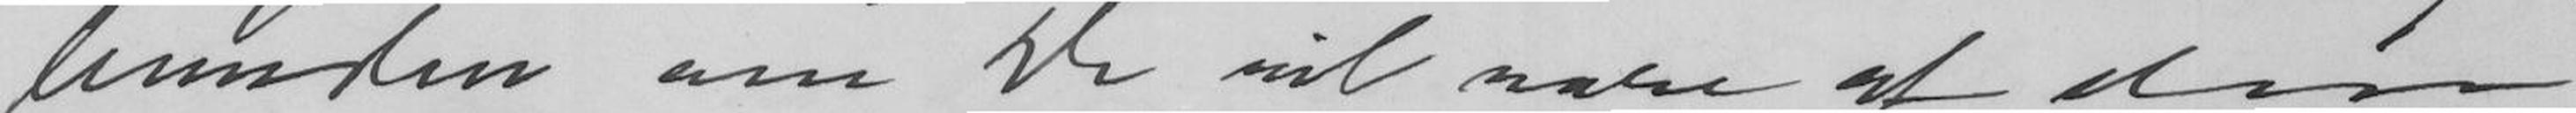bunden om De vil være af den
